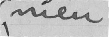, men
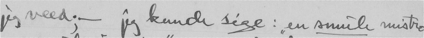jeg veed - jeg kunde sige: "en smule mistro
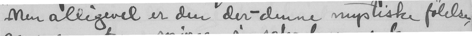Men alligevel er den der - denne mystiske følelse,
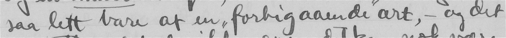saa lett bare af en "forbigaaende" art, - og det
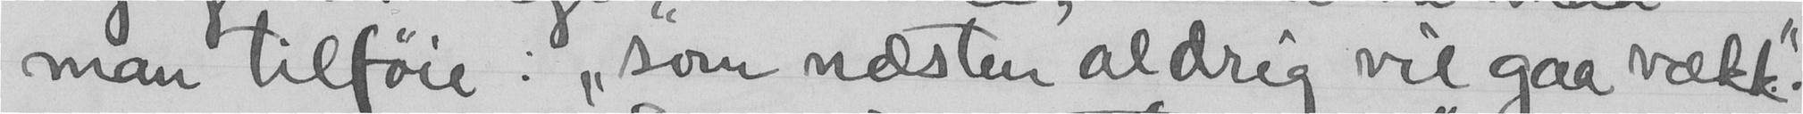man tilføie: "som næsten aldrig vil gaa vækk".
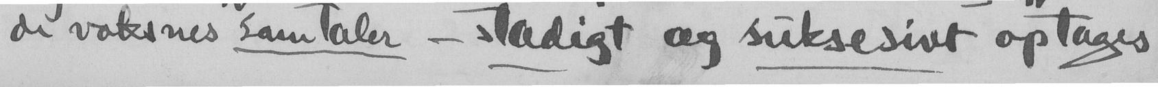de voksnes samtaler - stadigt og suksesivt optages
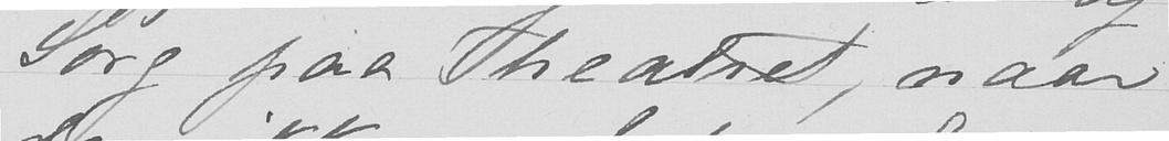Sorg paa Theatret, naar
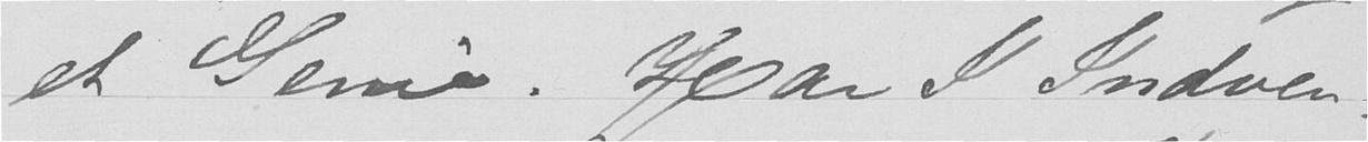et Genie. Har I Indven-
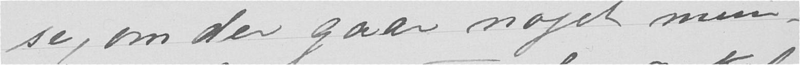se, om der gaar noget mun-
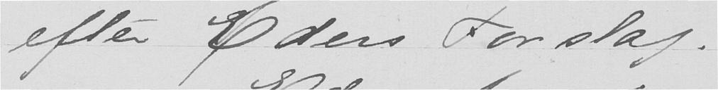efter Eders Forslag.
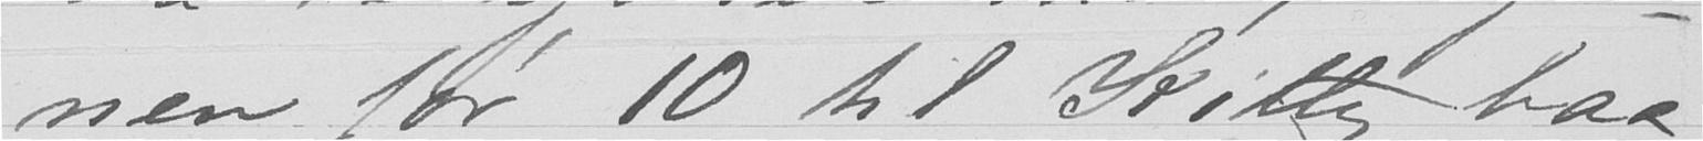nen før 10 til Kitty baa
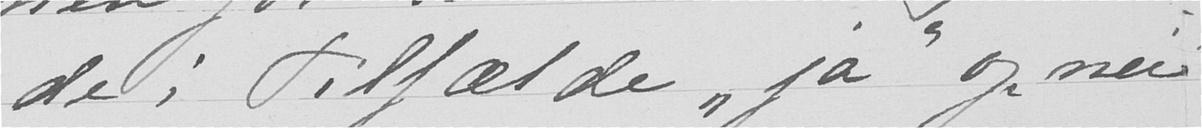de i Tilfælde "ja"  og "nei"
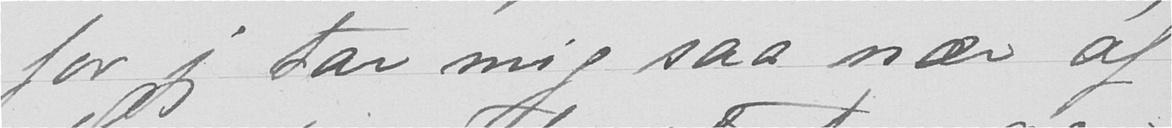for jeg tar mig saa nær af
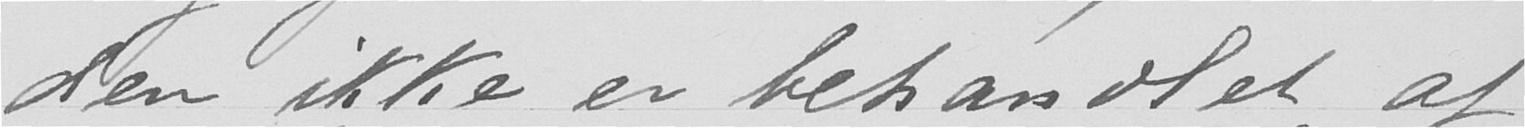den ikke er behandlet af
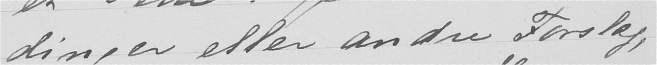dinger eller andre Forslag,
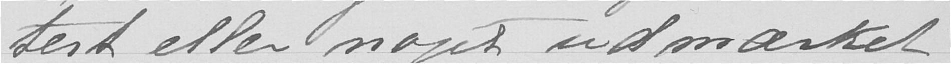tert eller noget udmærket,
